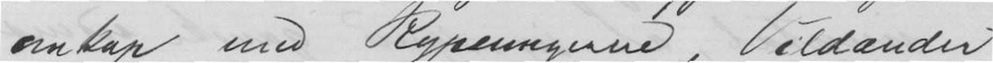omkap med Rypeungerne, Vildander
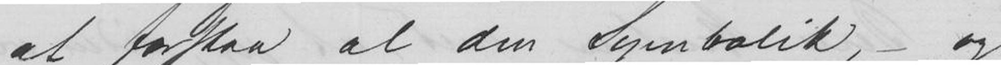at forstaa al den Symbolik, - og
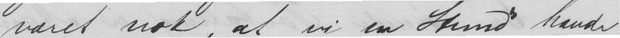været nok, at vi en Stund havde
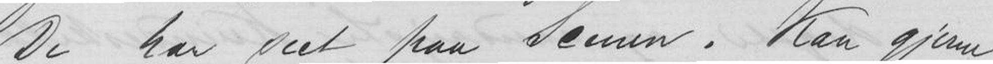De har seet paa Scenen. Kan gjerne
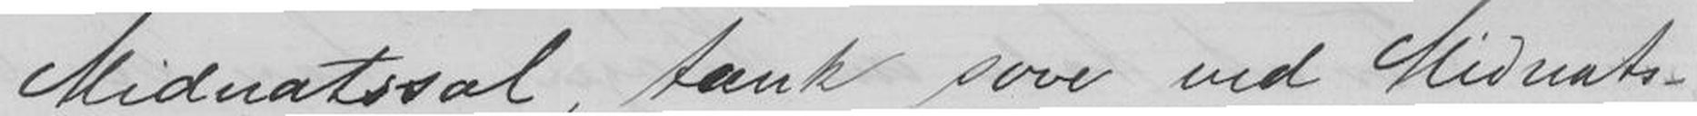Midnatssol, tænk sove ved Midnats-
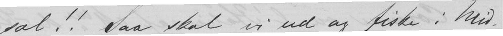sol!! Saa skal vi ud og fiske i Mid-
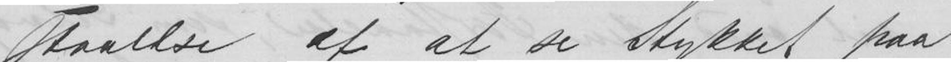staalse af at se stykket paa
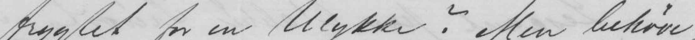frygtet for en Ulykke? Men behøve-
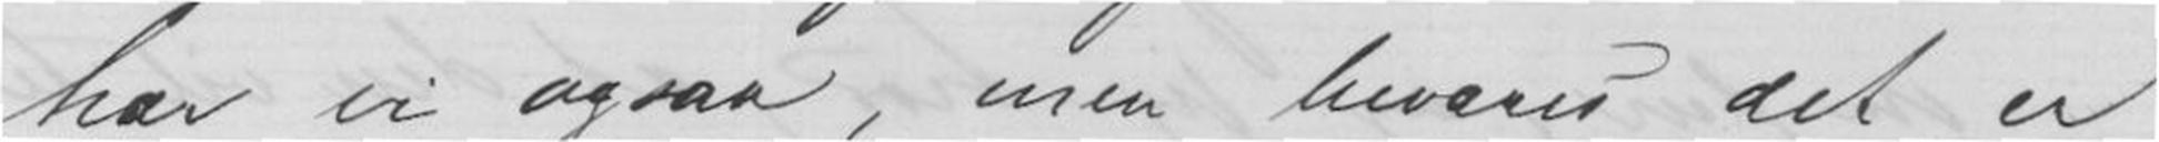har vi ogsaa, men bevares det er
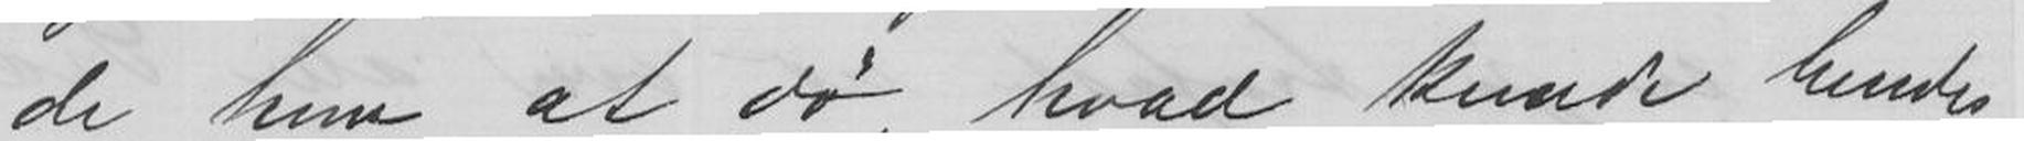de hun at dø, hvad kunde hendes
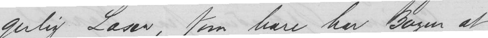gerlig Lærer, som bare har Bogen
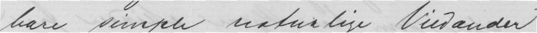bare simple naturlige Vildander
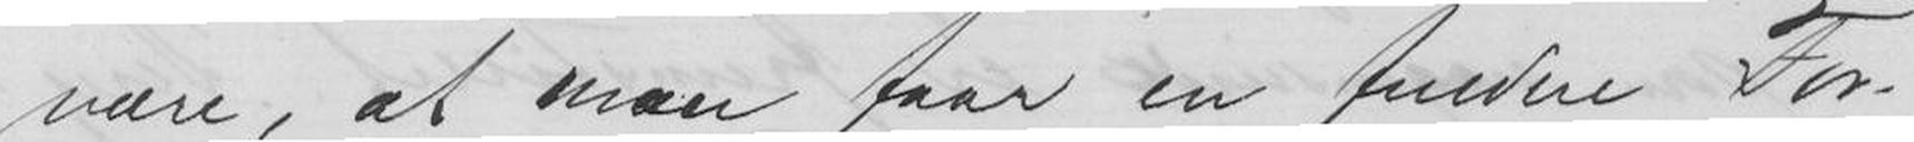være, at man faar en fuldere For-
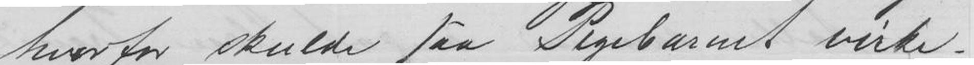hvorfor skulde saa Pigebarnet virke-
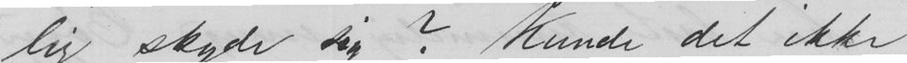lig skyde sig? Kunde det ikke
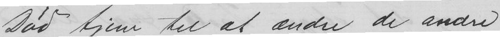Død tjene til at ændre de andre
